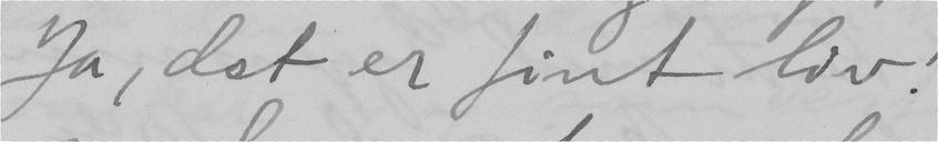Ja, det er fint liv!
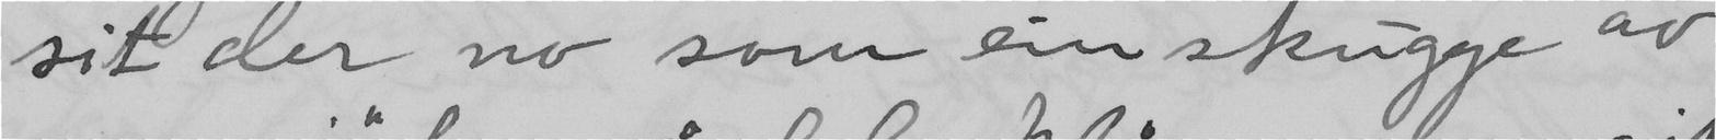sit der no som ein skugge av
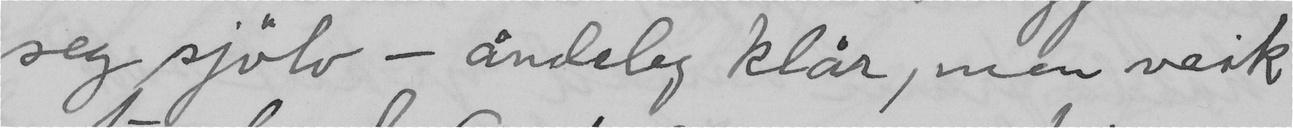seg sjölv – åndeleg klår, men veik
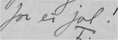for ei jol!
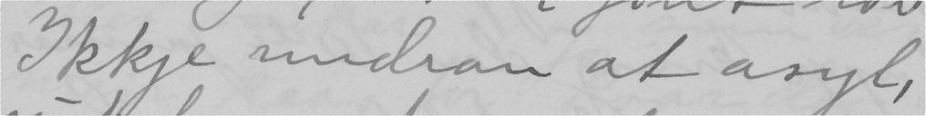Ikkje undran at asyl,
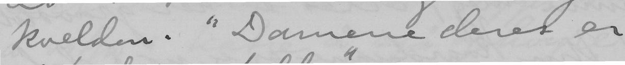kvelden. "Damene deres er
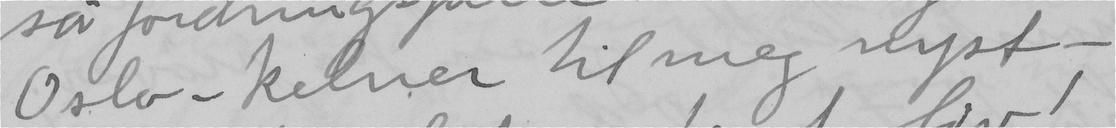Oslo-kelner til meg nyst –
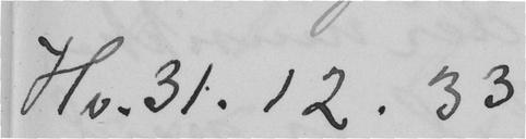Hv. 31.12.33
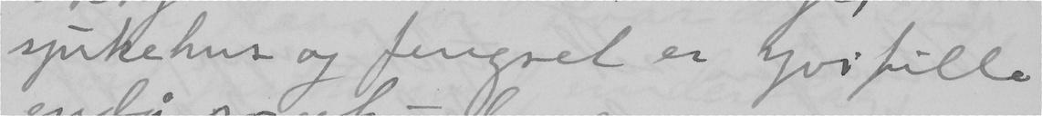sjukehus og fengsel er yvifulle
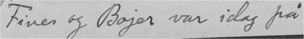Fines og Bojer var idag på
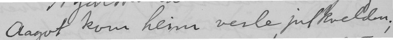Aagot kom heim vesle julkvelden;
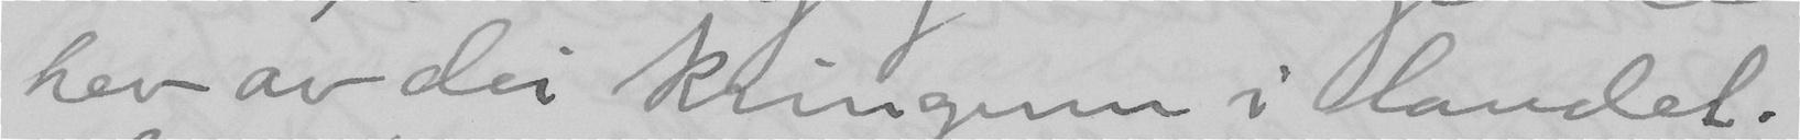hev av dei kringum i landet.
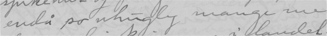endå so uhugleg mange me
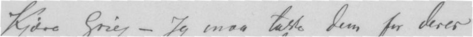Kjære Grieg - Jeg maa takke Dem for Deres
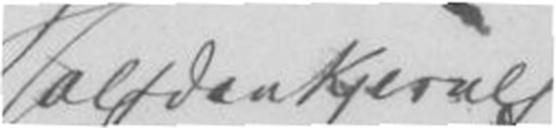Halfdan Kjerulf
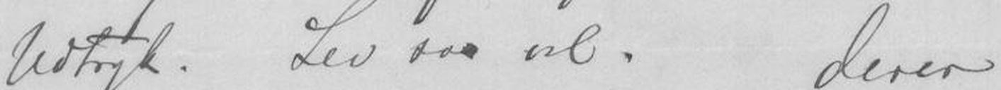Udtryk. Lev sa vel. Deres
